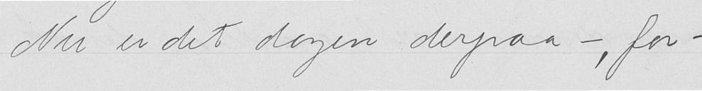Nu er det dagen derpaa -, for-
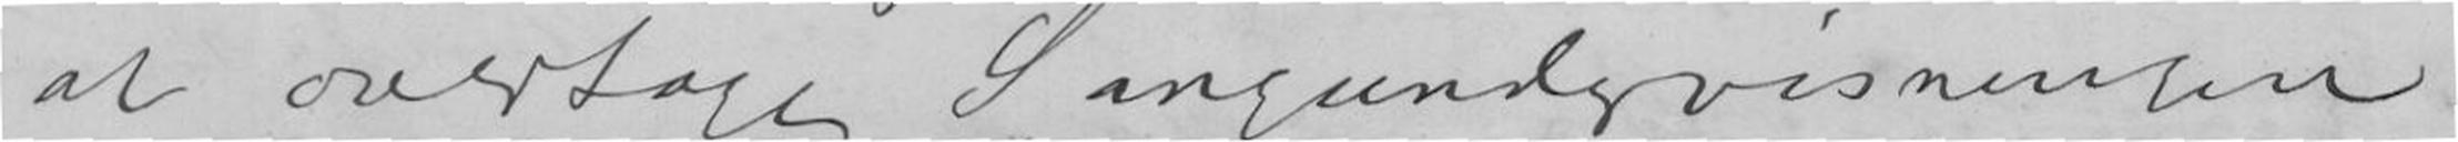at overtage Sangundervisningen
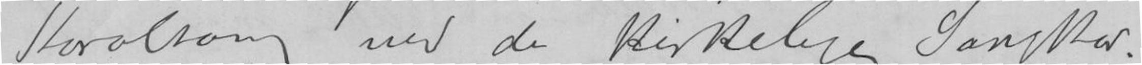i Koralsang ved de kirkelige Sangkor.
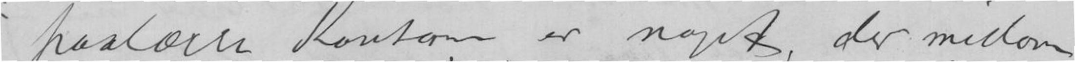paalægge Kantorne er noget, der mellom
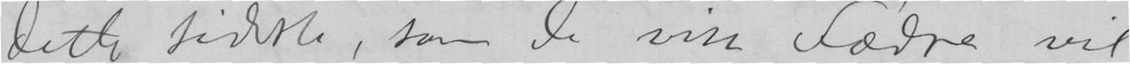Dette sidste, som De vise Fædre vil
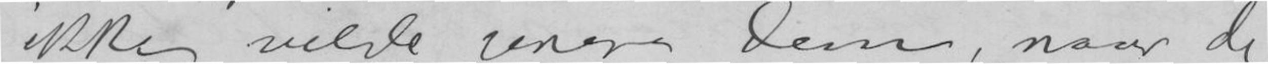ikke vilde genere Dem, naar de
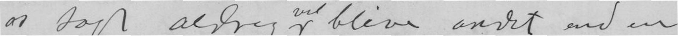os sagt aldrig vil blive andet end en
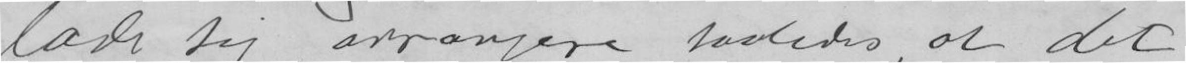lade sig arrangere saaledes, at det
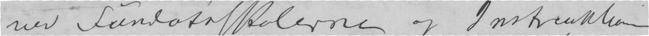ved Fundotskolerne og Instruktionen
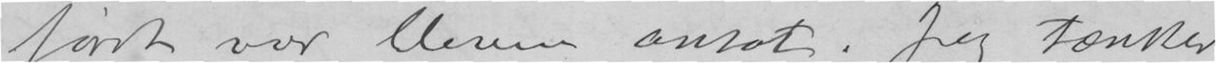først var bleven ansat. Jeg tænker
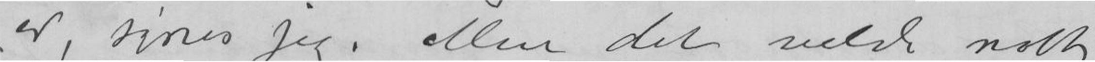er, synes jeg. Men det vilde nok
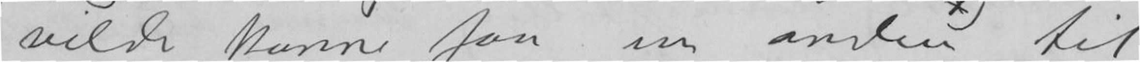vilde kunne faa en anden*) til
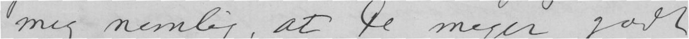mig nemlig, at De meget godt
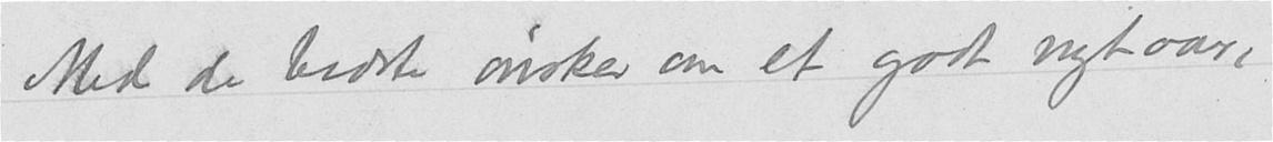Med de bedste ønsker om et godt nytaar,
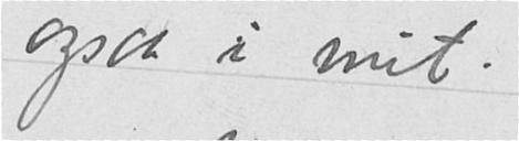ogsaa i mit.
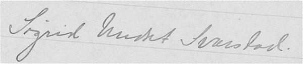Sigrid Undset Svarstad.
